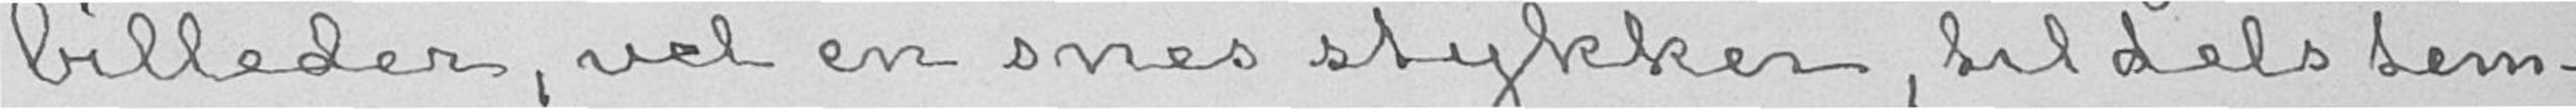billeder, vel en snes stykker, tildels tem-
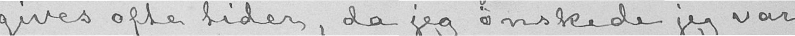gives ofte tider, da jeg ønskede jeg var
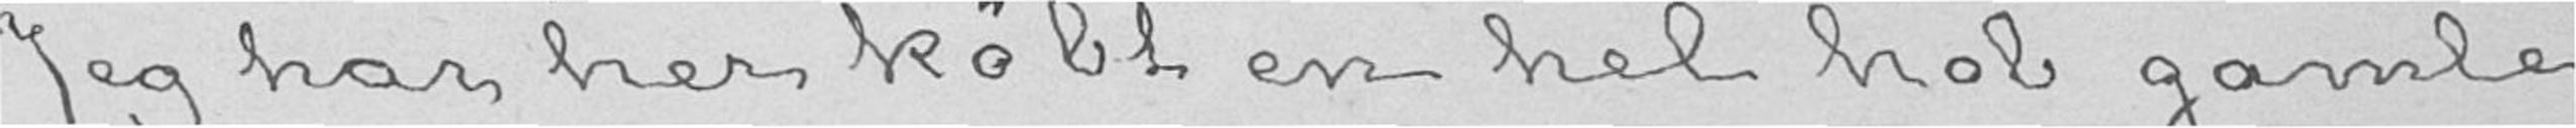Jeg har her købt en hel hob gamle
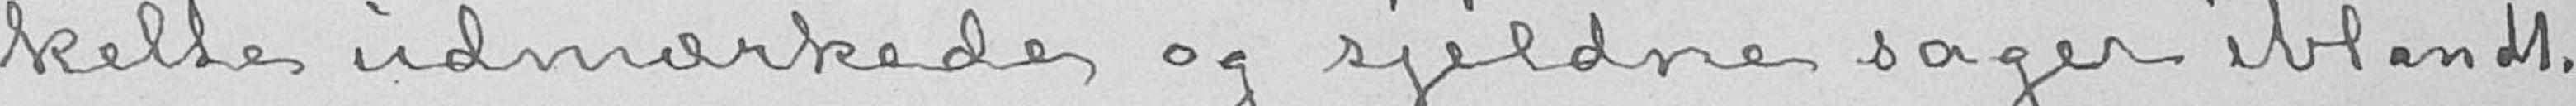kelte udmærkede og sjeldne sager iblandt.
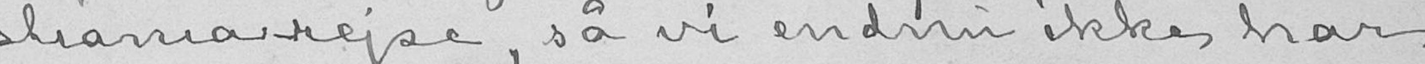stianiarejse, så vi endnu ikke har
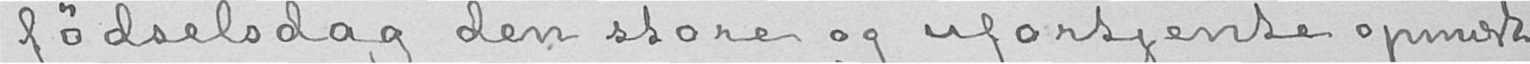fødselsdag den store og ufortjente opmærk-
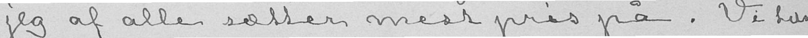jeg af alle sætter mest pris på. Vi tæn-
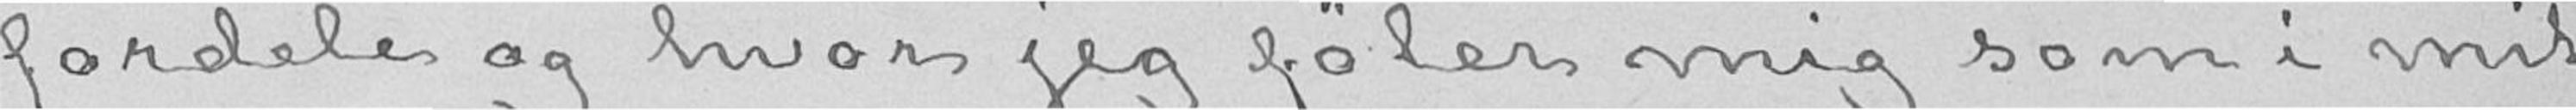fordele, og hvor jeg føler mig som i mit
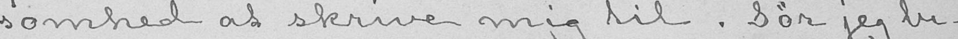somhed at skrive mig til. Tør jeg be-
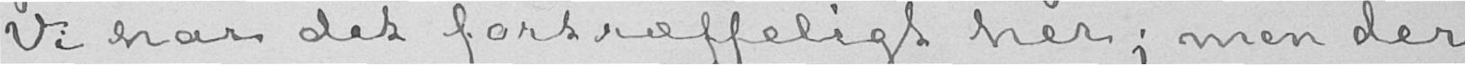Vi har det fortræffeligt her; men der
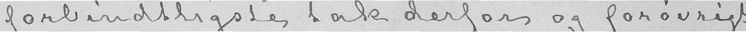forbindtligste tak derfor og forøvrigt
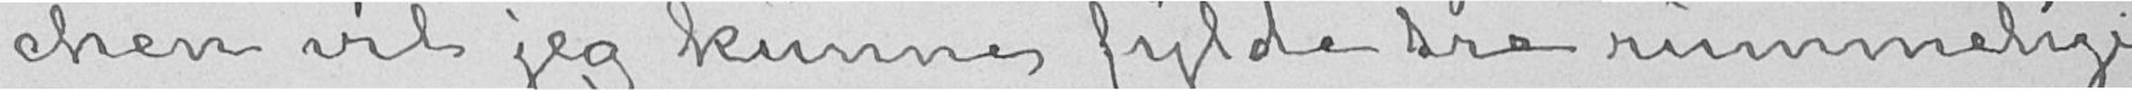chen vil jeg kunne fylde tre rummelige
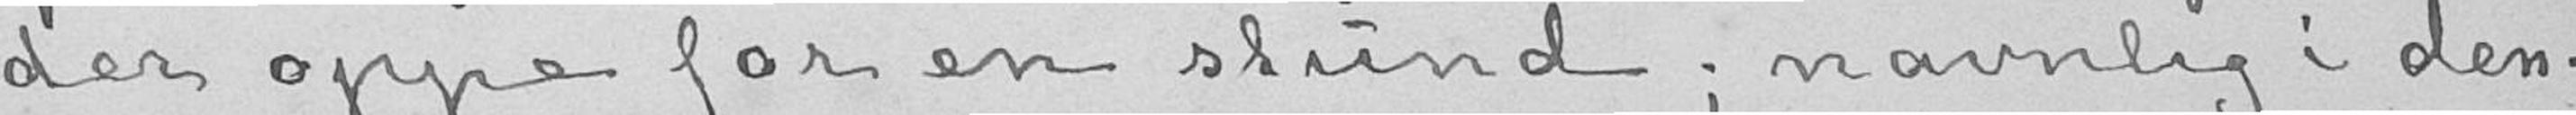der oppe for en stund; navnlig i den-
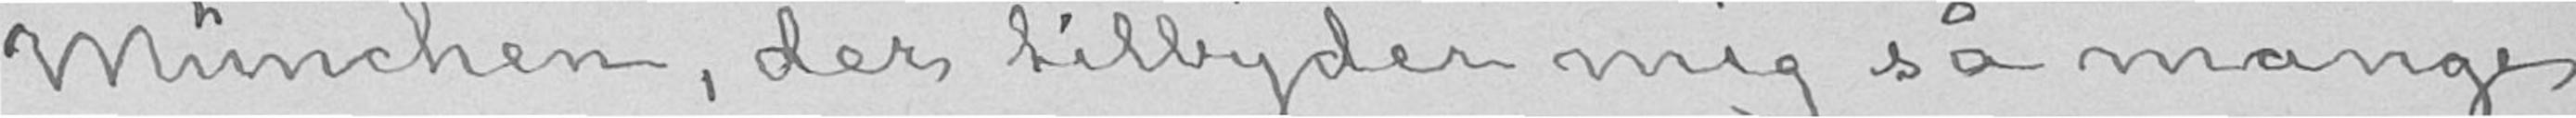München, der tilbyder mig så mange
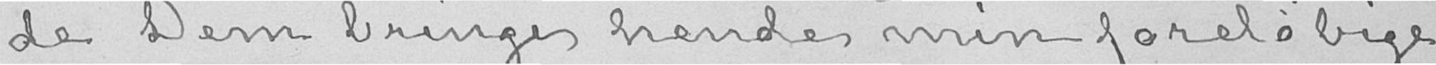de Dem bringe hende min foreløbige
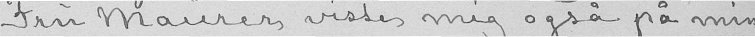Fru Maurer viste mig også på min
